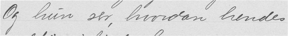Og hun ser, hvordan hendes
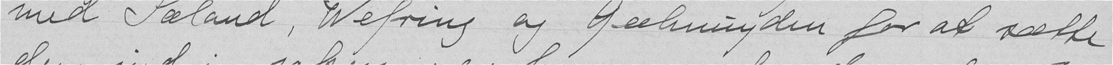med Sæland, Wefring og Geehmyden for at sætte
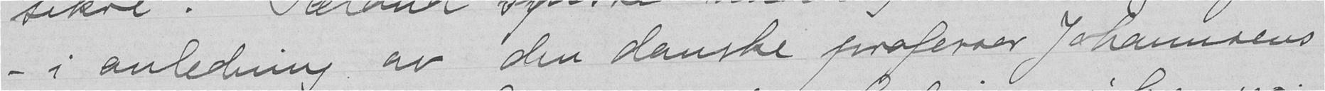- i anledning av den danske profesoer Johannsens
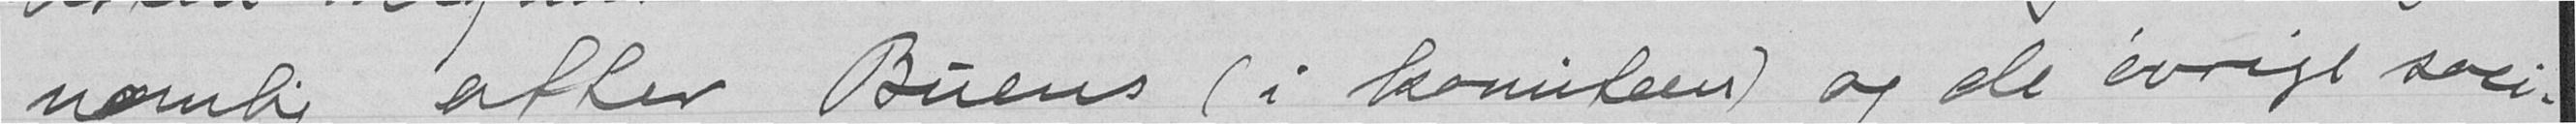næmnlig atter Buens (i komiteen) og ele øvrigt soci
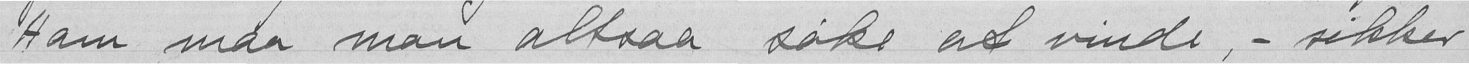Ham maa man altsaa søke at vinde, - sikker
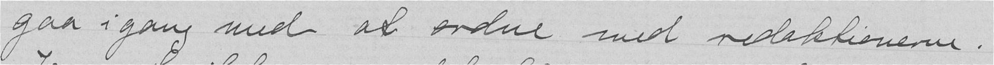gaa i gang med at ordne med redaktionerne.
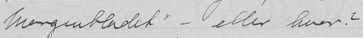"Morgenbladet" - eller hvor?
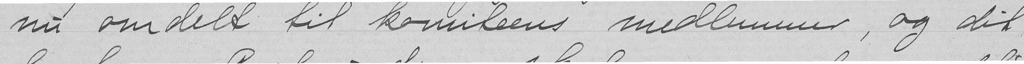nu omdelt til komiteens medlemmer, og dit
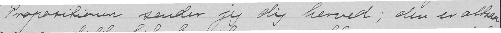Propositionen sender jeg dig herved; den er alfran
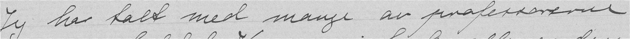Jeg har faet med mange av professorerne
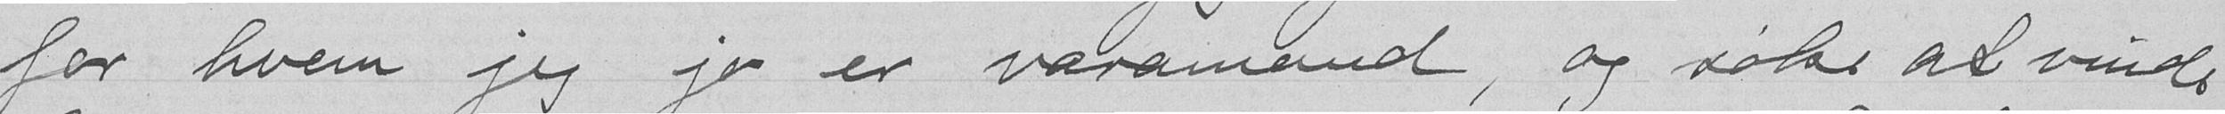for hvem jeg jo er varamand, og søke at vinde
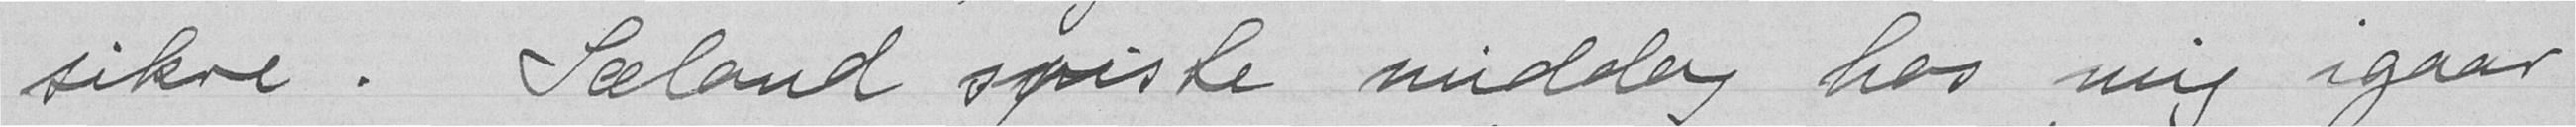sikre. Sæland spiste middag hos mig igaar
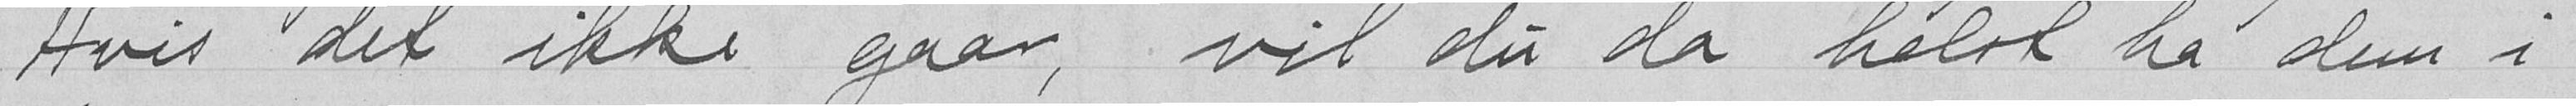Hvis det ikke gaar, vil du da helst fa dem i
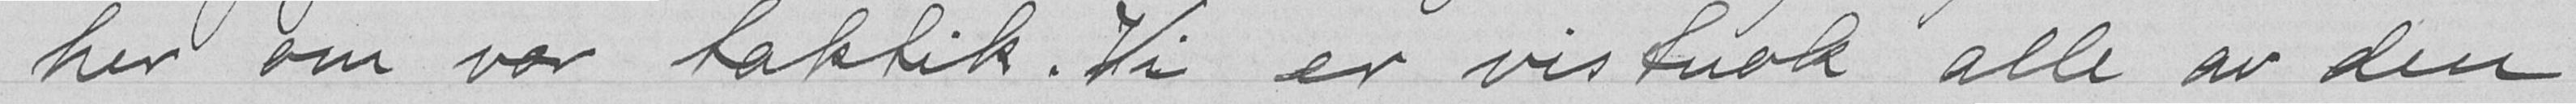her om var taktik. Vi er vistnok alle av den
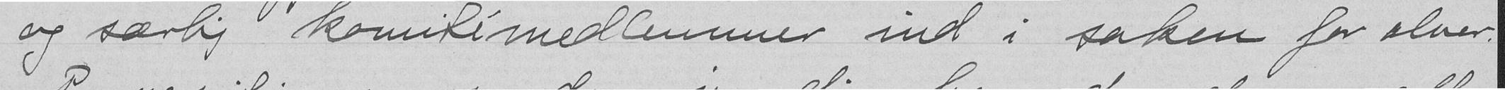og særlig komitémedlemmer ind i saken for alvor.
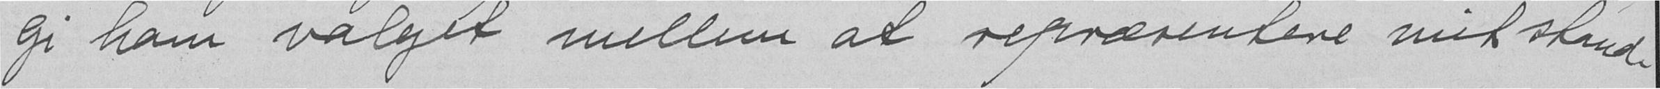- gi ham valget mellem at repræsentere mit stand
